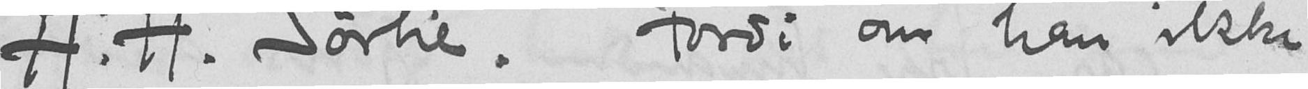A.H. Sørlie. Fordi om han ikke
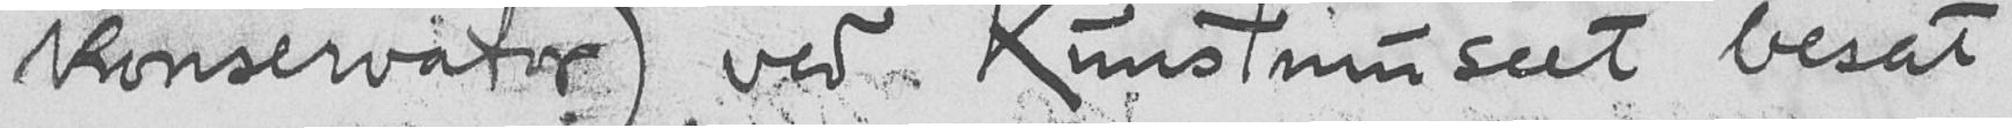konservator) ved Kunstmuseet besat
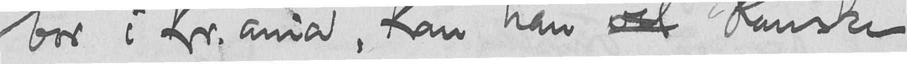bor i Kr.ania, kan han kanske
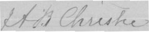JAB Christie
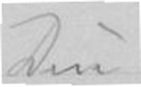Din
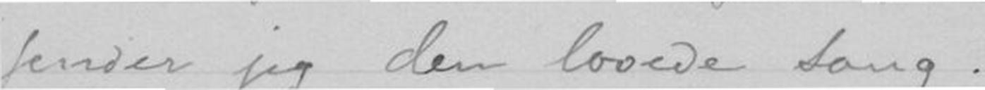sender jeg den lovede sang.
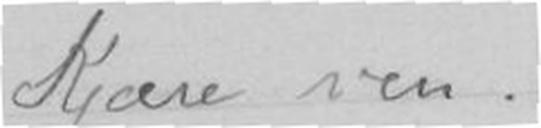Kjære ven.
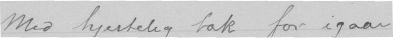Med hjertelig tak for igaar
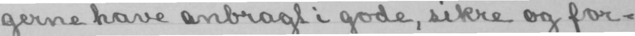gerne have anbragt i gode, sikre og for-
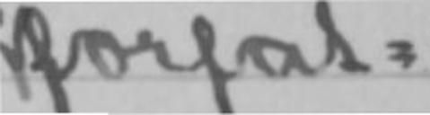forfat-
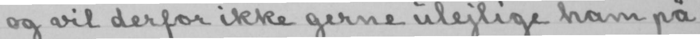og vil derfor ikke gerne ulejlige ham på
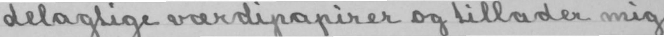delagtige værdipapirer og tillader mig
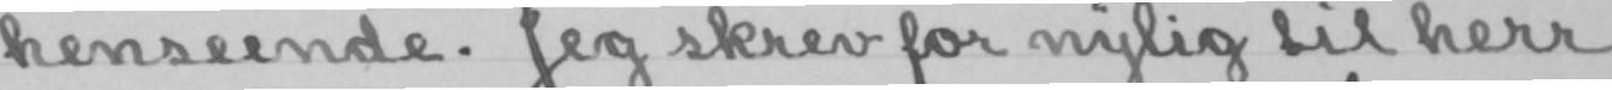henseende. Jeg skrev for nylig til herr
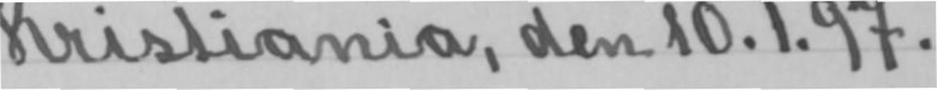Kristiania, den 10.1.97.
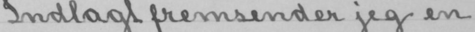Indlagt fremsender jeg en
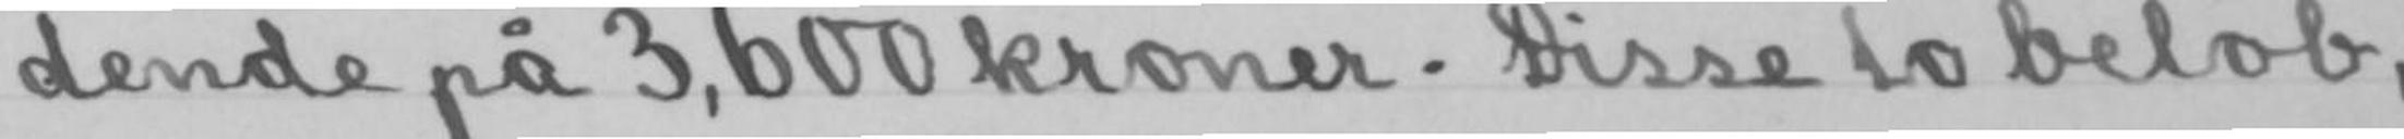dende på 3,600 kroner. Disse to beløb,
